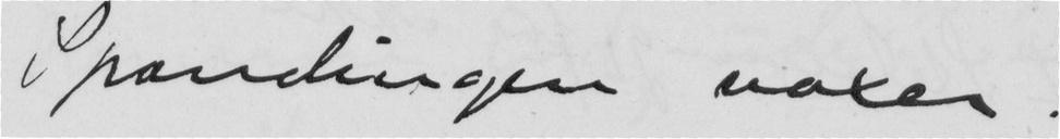Spændingen voxer.
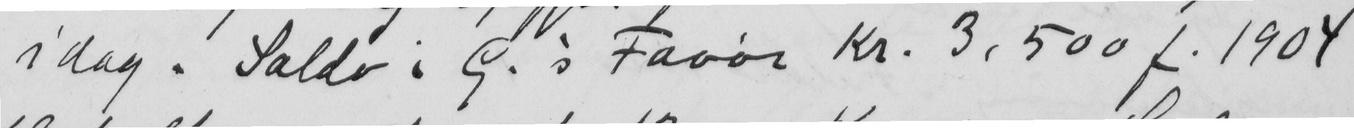idag. Saldo i G.'s Favør Kr. 3,500 f. 1904
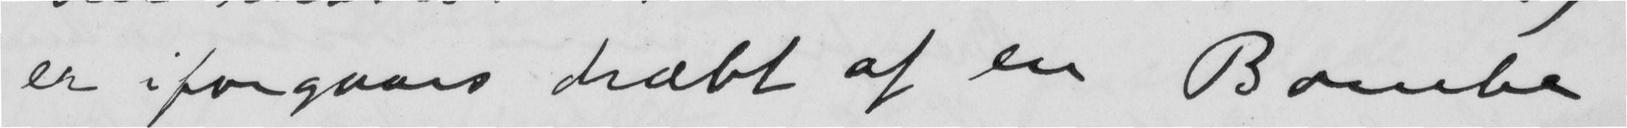er iforgaars dræbt af en Bombe
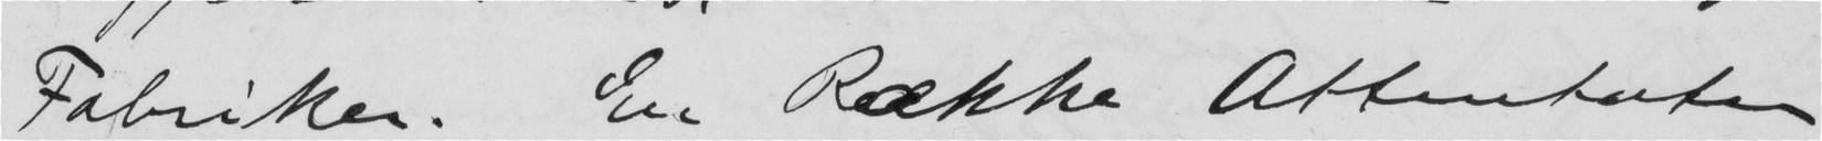Fabriker. En Ræække Attentater
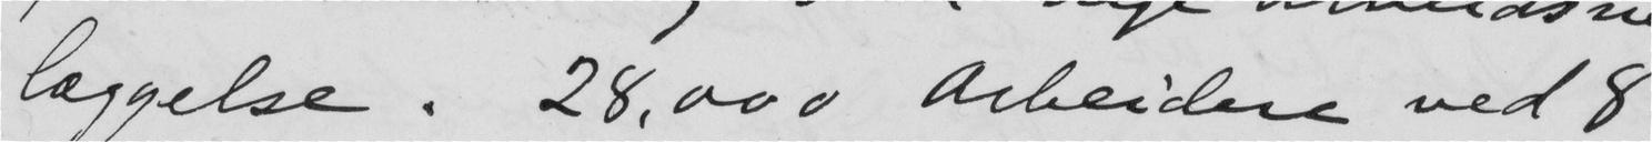læggelse. 28,000 Arbeidere ved 8
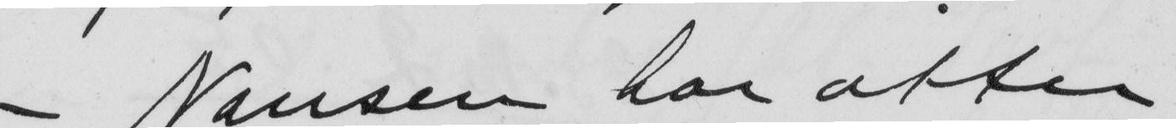- Nansen har atter
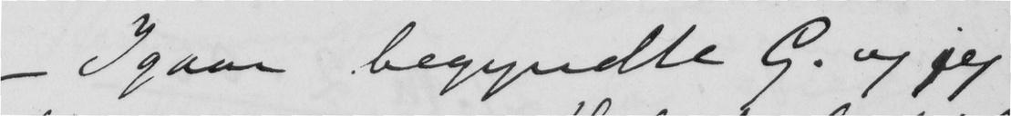– Igaar begyndte G. og jeg
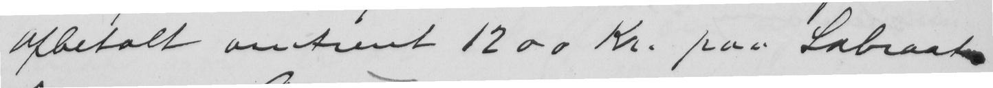afbetalt omtrent 1200 Kr. paa Labraat
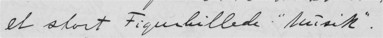et stort Figurbillede. "Musik".
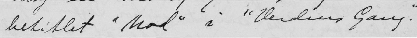betitlet "Mod" i "Verdens Gang.
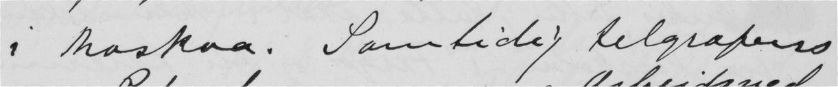i Moskva. Samtidig telgraferes
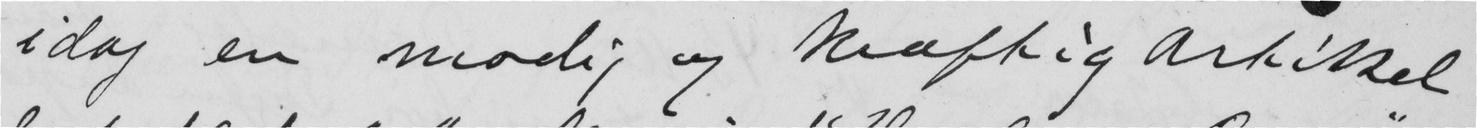idag en modig og kraftig Artikel
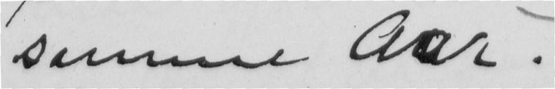samme Aar.
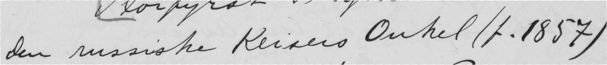den russiske Keisers Onkel (f. 1857)
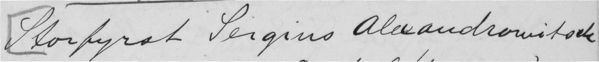Storfyrst Sergins Alexandrovitsch
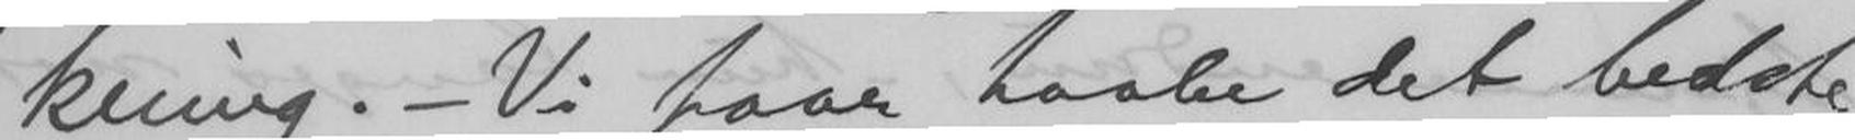kring. - Vi faar haabe det bedste
